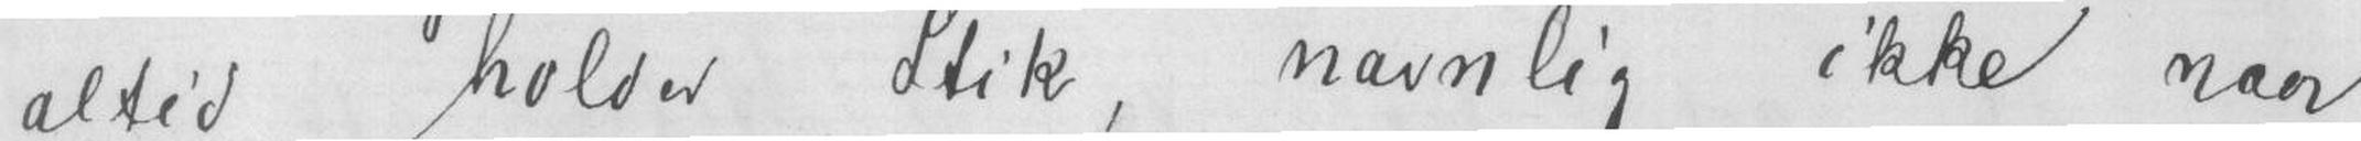altid holder Stik, navnlig ikke, naar
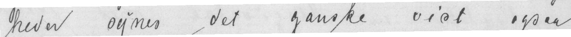heder synes det ganske vist ogsaa
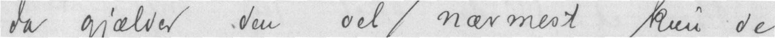da gjælder den vel nærmest kun de
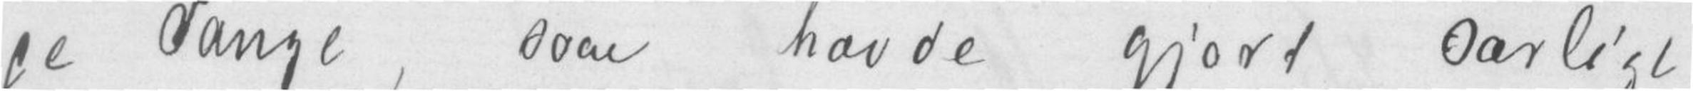de Sange, som havde gjort narlige
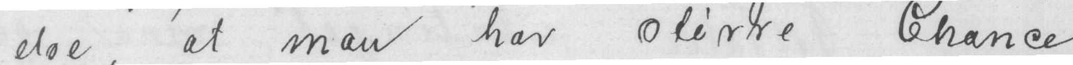else, at man har større Chance
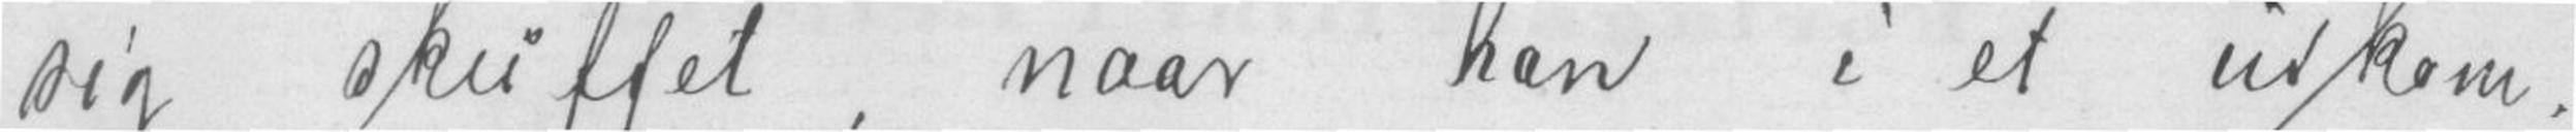sig skuffet, naar han i et udkom-
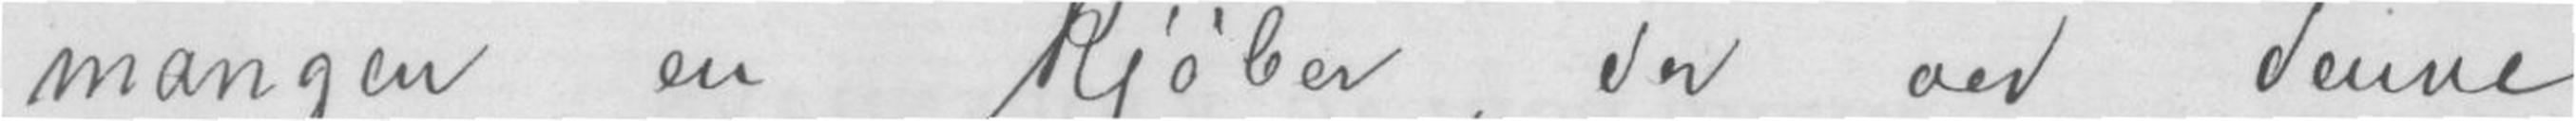mangen en Kjøber, der ved denne
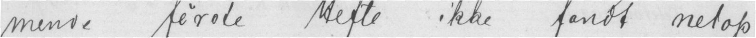mende første Hefte ikke fandt netop
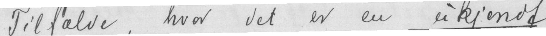Tilfælde, hvor det er en ukjendt
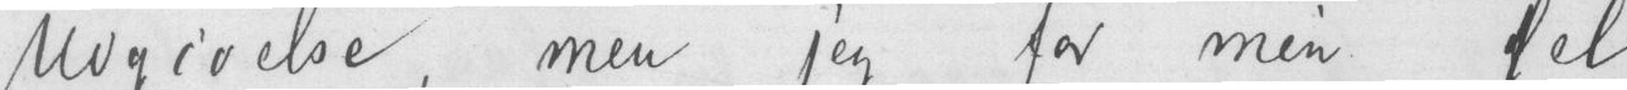Udgivelse, men jeg for min del
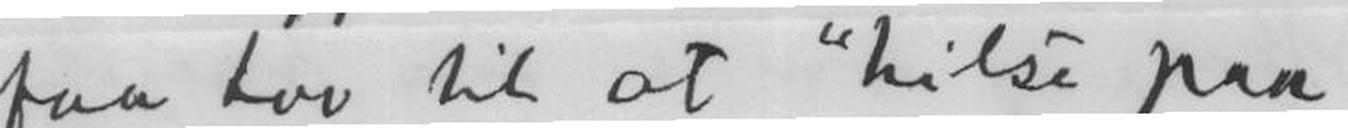faa lov til at "hilse paa
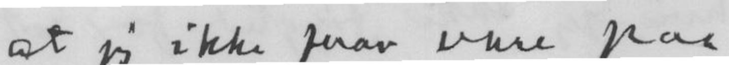at jeg ikke faar være paa
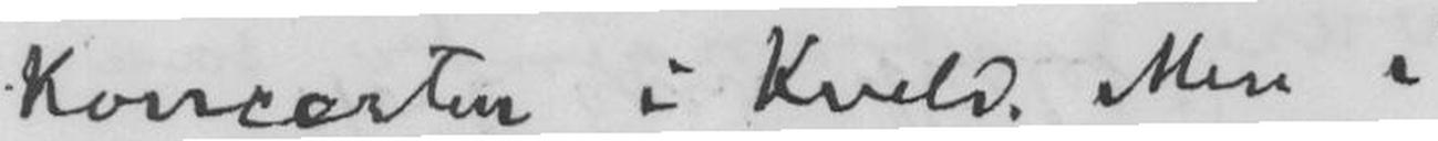Koncerten i Kveld. Men i
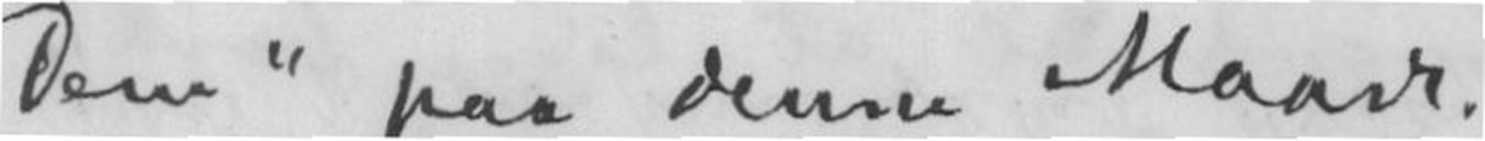Dem" paa denne Maade.
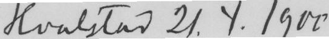Hvalstad 21.4.1900
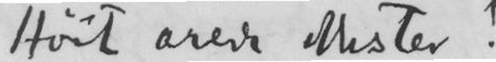Høit ærede Mester!
